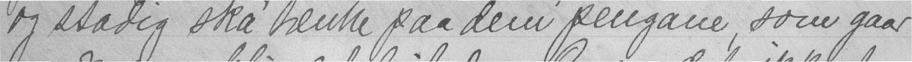og stadig ska' tenke paa dem pengane, som gaar
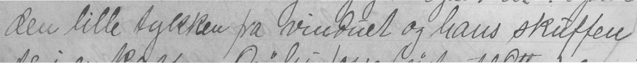den lille tykken fra vinduet og hans skuffen
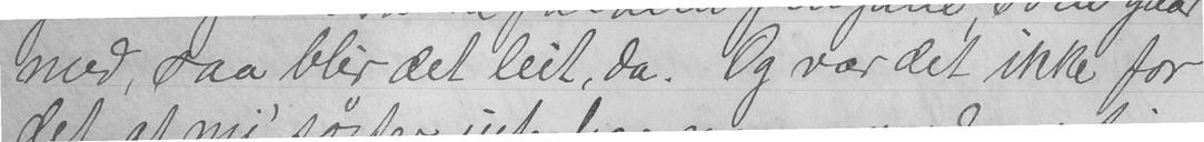med, saa blir det leit, da. Og var det ikke for
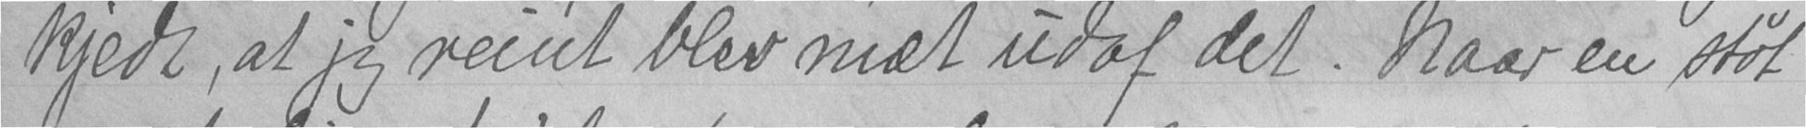kjedt, at jeg reint blev mæt udaf det. Naar en støt
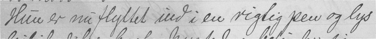Hun er nu flyttet ind i en rigtig pen og lys
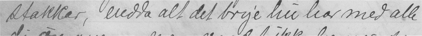stakkar, endda alt det bry'e hu har med alle
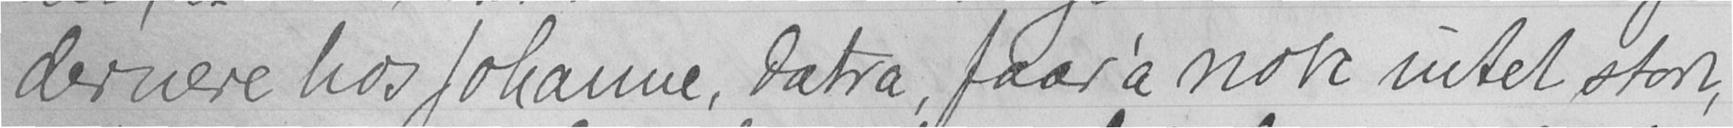dernere hos Johanne, datra, faar'a nok intet stort,
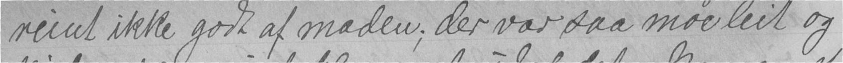reint ikke godt af maden; der var saa møe leit og
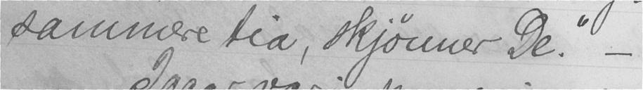sammere tia, skjønner De." -
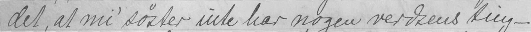det, at mi' søster inte har nogen verdsens ting -
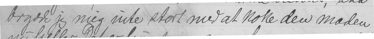brydde jeg mig inte stort med at koke den maden
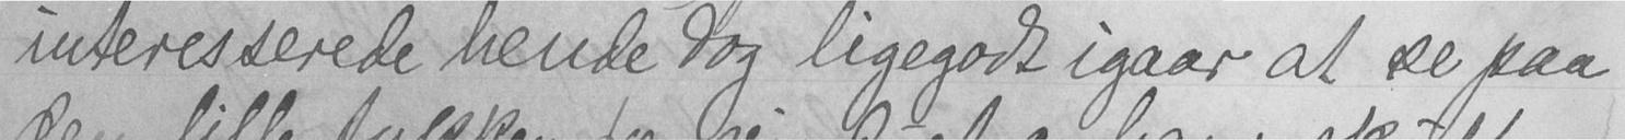interesserede hende dog ligegodt igaar at se paa
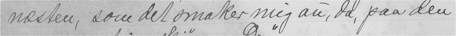næsten, som det smaker mg au, da, paa den
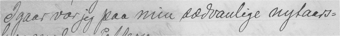I gaar var jeg paa min sædvanlige nytaars-
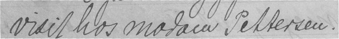visit hos madam Pettersen.
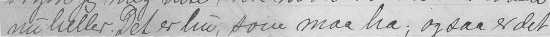nu heller. Det er hu, som maa ha; og saa er det
